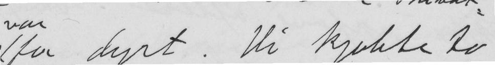for dyrt. Vi kjøbte to
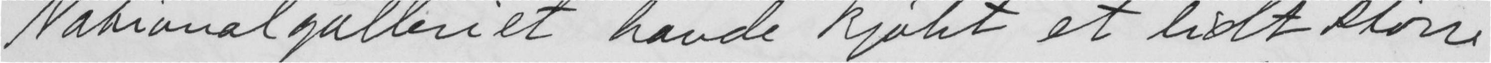Nationalgalleriet havde kjøbt et lidt større
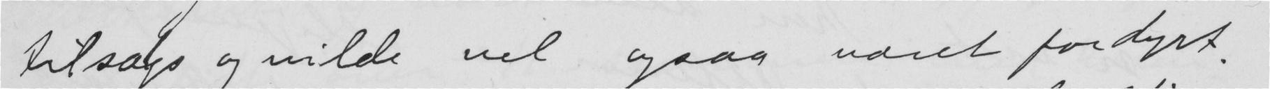tilsalgs og vilde vel ogsaa været fordyrt.
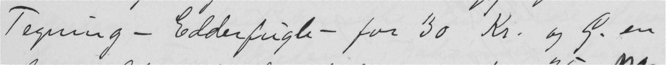Tegning - Edderfugle - for 30 Kr. og G. en
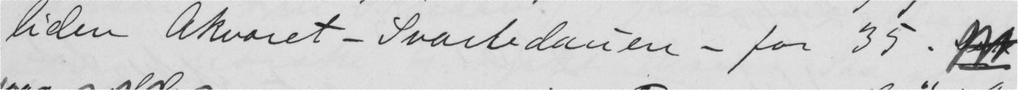liden Akvaret - Svartedauen - for 35.
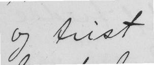og trist
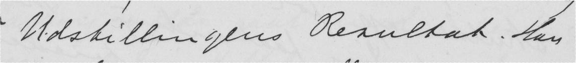Udstillingens Resultat. Han
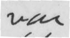var
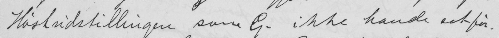Høstudstillingen som G. ikke havde set før.
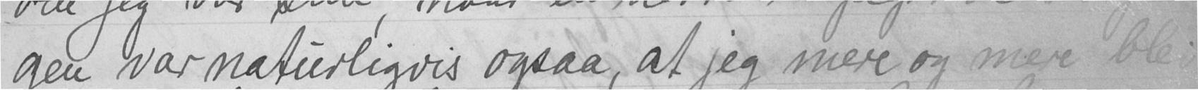gen var naturligvis ogsaa, at jeg mere og mere ble
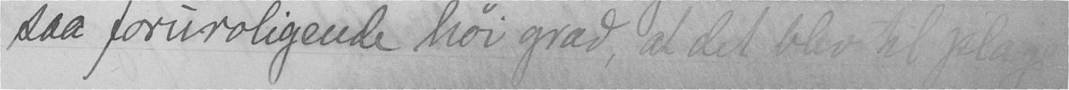saa foruroligende høi grad at det blev til plage
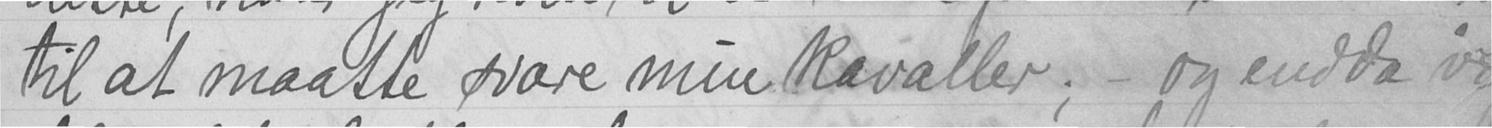til at maatte svare min kavaller; - og endda vi
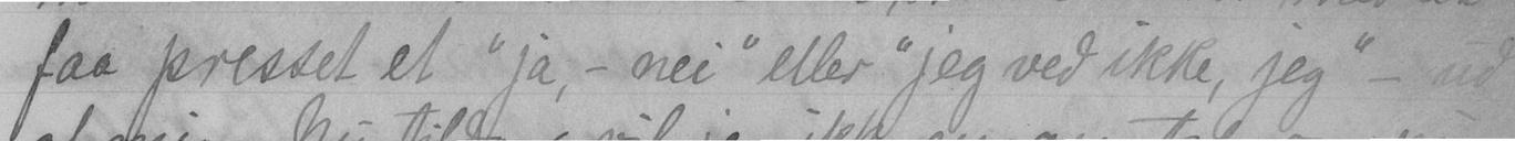faa presset et "ja, - nei", eller "jeg ved ikke, jeg" - ud
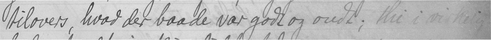tilovers, hvad der baade var godt og ondt; thi i virkelig-
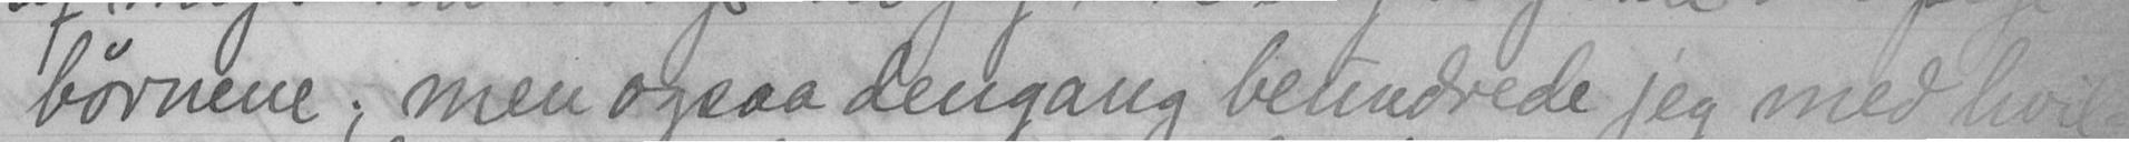børnene; men ogsaa dengang beundrede jeg med hvil-
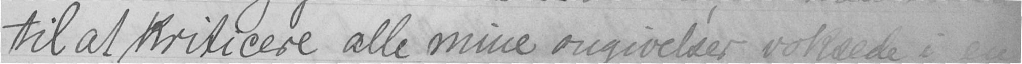til at kriticere alle mine ongivelser voksede i en
